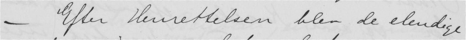- Efter Henrettelsen blev de elendige
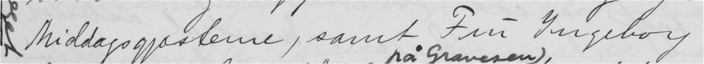Middagsgjæsterne, samt Fru Ingeborg
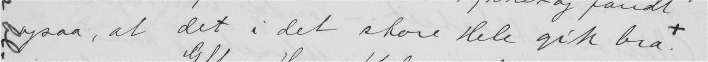ogsaa, at det i det store Hele gik bra. x
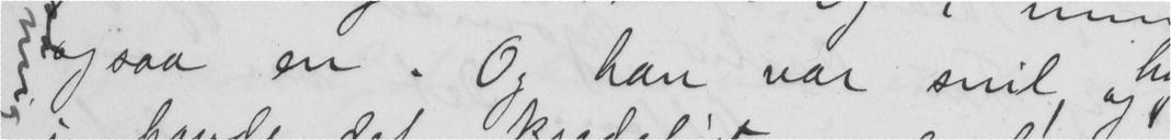ogsaa en. Og han var snil, og
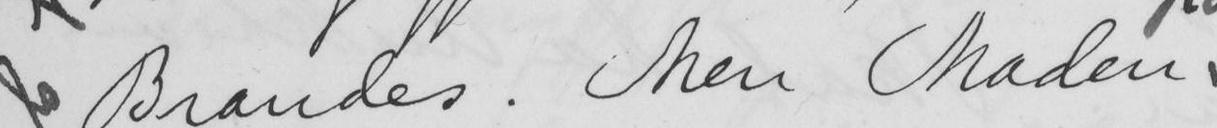Brandes. Men Maden
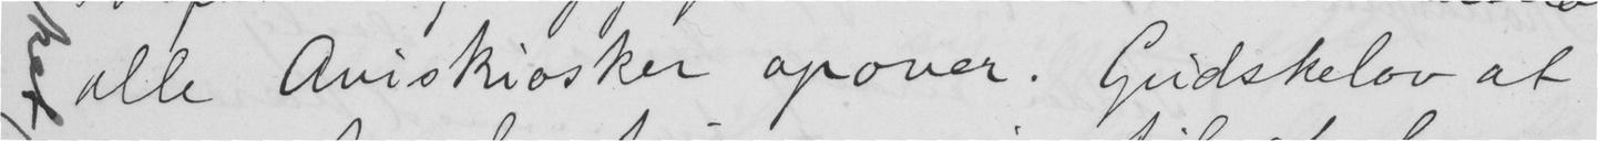alle Aviskiosker opover. Gudskelov at
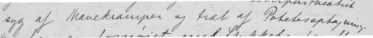syg af Mavekramper og træt af Potetesoptagning.
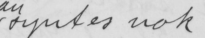syntes nok
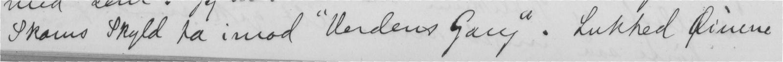Skams Skyld ta imod "Verdens Gang". Lukhed Øinene
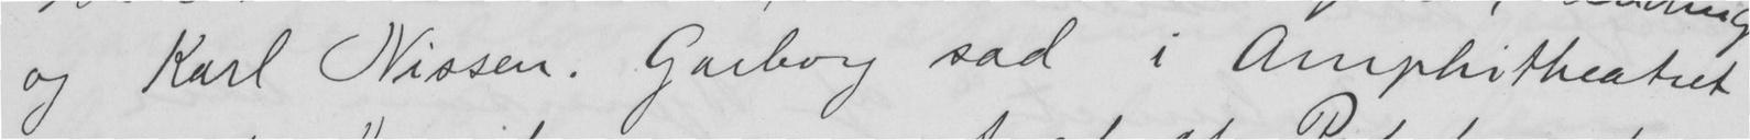og Karl Nissen. Garborg sad i Amphitheatret
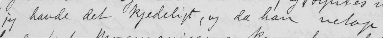jeg havde det kjedeligt, og da han netop
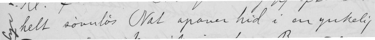helt søvnløs Nat opover hid i en ynkelig
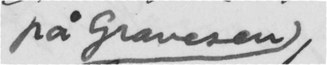på Gravesen
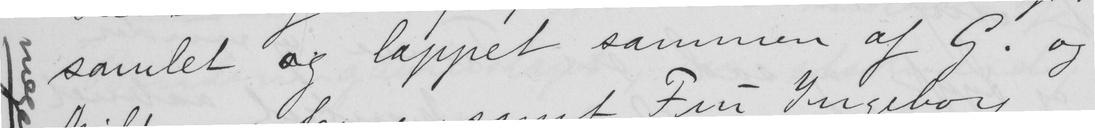samlet og lappet sammen af G. og
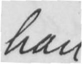han
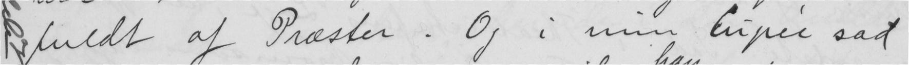fuldt af Præster. Op i min Cupeé sad
